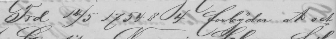Frd 14/5 1754 § 4 forbyder at set-
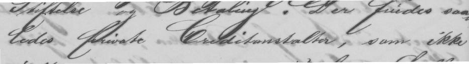ledes private Creditoranstalter, som ikke
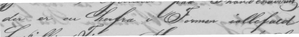der er en herfra i Formen iallefald
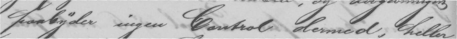paabyder ingen Control dermed, heller
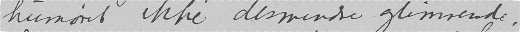humøret ikke desmindre glimrende, han ligger 4 timer i træk ute i haven
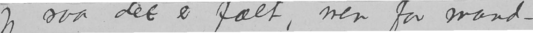jeg saa det er fælt, men for mand-
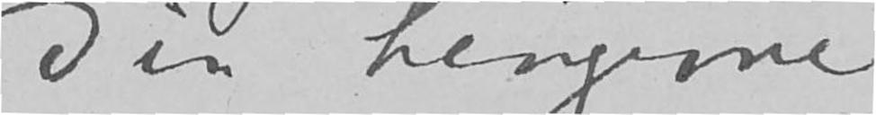Din hengivne
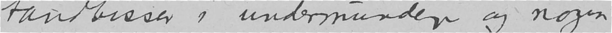tandbisser i undermunden og nogen
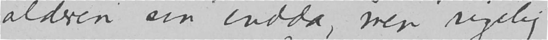alderen sin endda, men rigelig
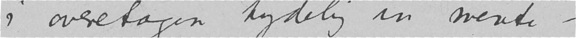i overetagen tydelig in mente –
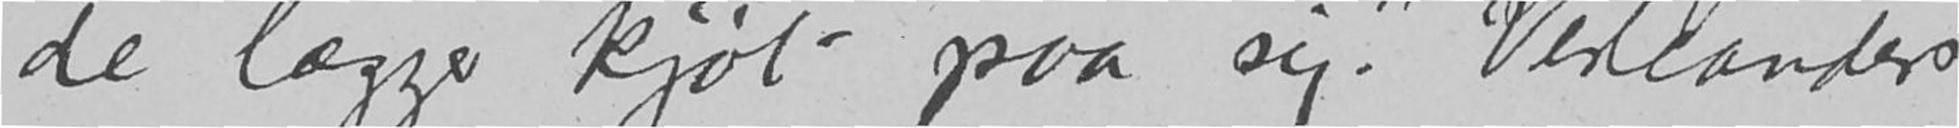de lægger kjøt paa sig. Vesleanders
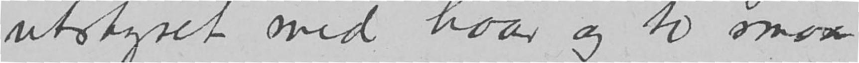utstyret med haar og to smaa
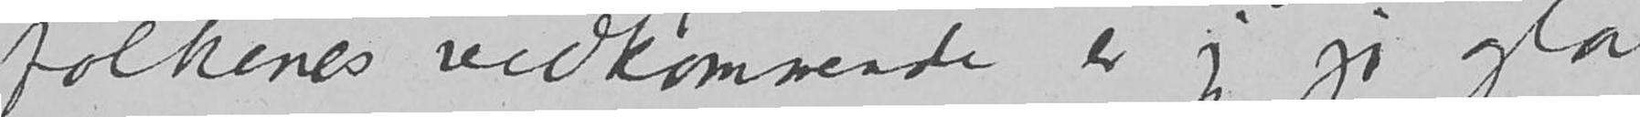folkenes vedkommende er jeg jo gla
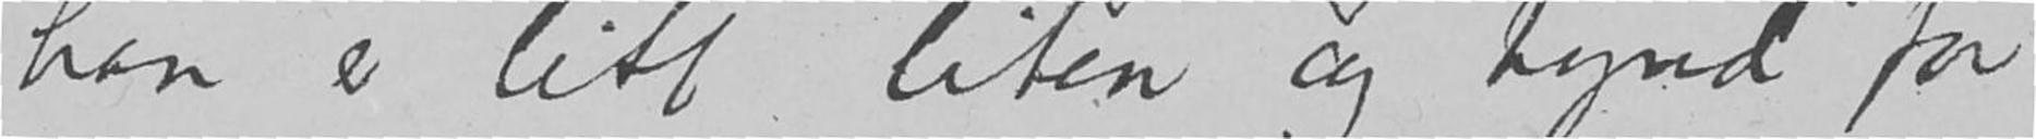han er litt liten og tynd for
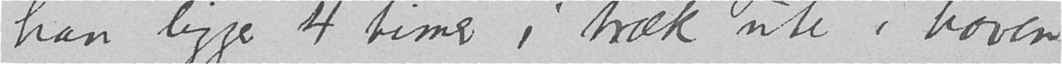han ligger 4 timer i træk ute i haven
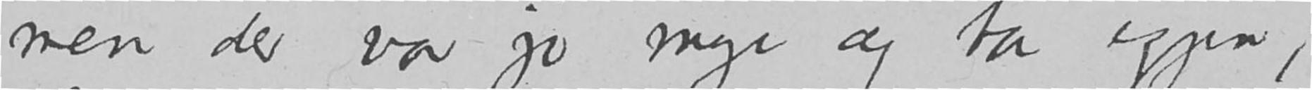men der var jo mye at ta igjen;
